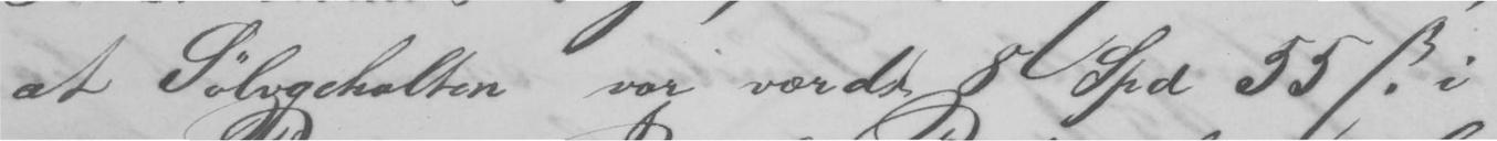at Sølvgehalten var værdt 8 Spd 55 ß. i
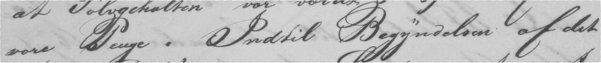vore Penge. Indtil Begyndelsen af det
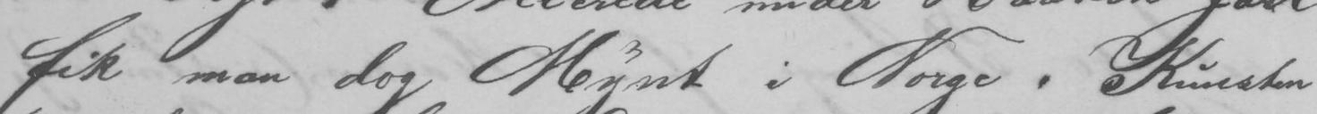fik man dog Mynt i Norge. Kunsten
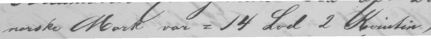norske Mark var = 14 Lod 2 Kvintin,
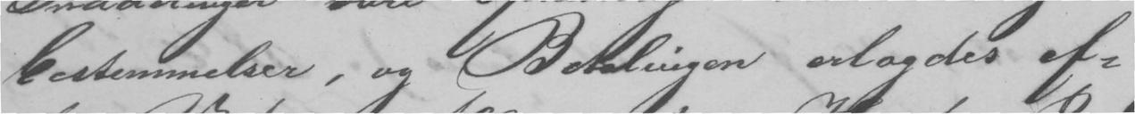bestemmelser, og Betalingen erlagdes ef-
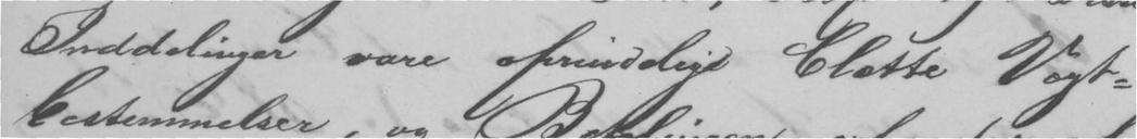Indelinger vare oprindelige blotte Vægt-
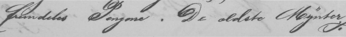fremdeles Pengene.  De ældste Mynter
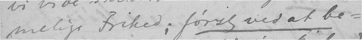melige Frihed; først ved at be-
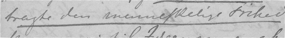tragte den menneskelige Frihed
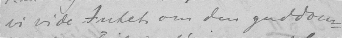vi vide Intet om den guddom-
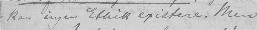kan ingen Ethik existere. Men
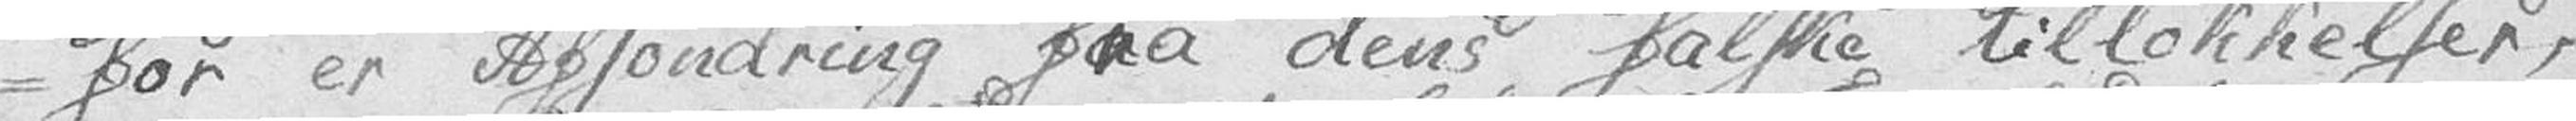-for er Afsondring fra dens falske tillokkelser,
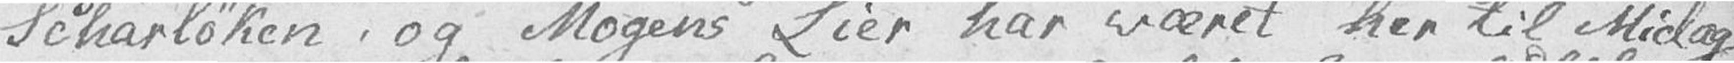Scharløken og Mogens Lier har været her til Midag
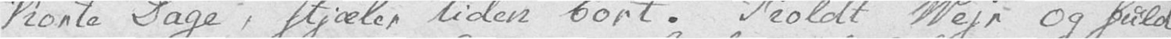korte Dage, stjæler tiden bort. Koldt Vejr og fuld
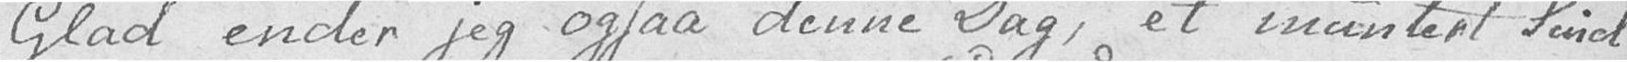Glad ender jeg ogsaa denne Dag, et muntert Sind
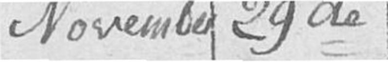November 29de
